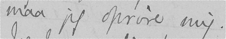maa jeg oprøre mig.
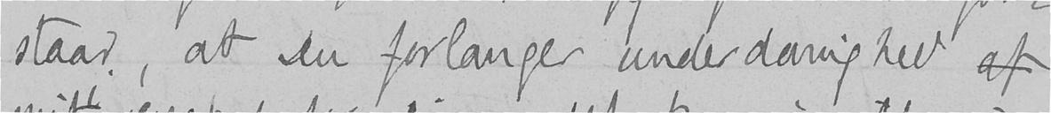staar, at Du forlanger underdanighed af
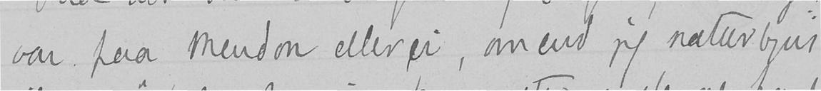var paa Mendon eller ei, om end jeg naturligvis
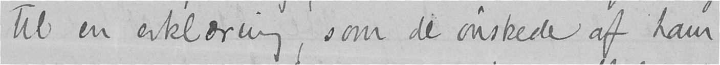til en erklæring, som de ønskede af ham,
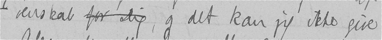venskab for Dig, og det kan jeg ikke give.
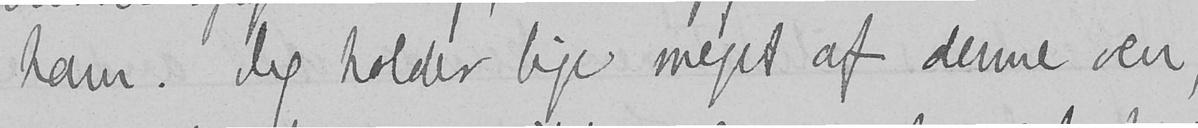ham. Jeg holder lige meget af denne ven,
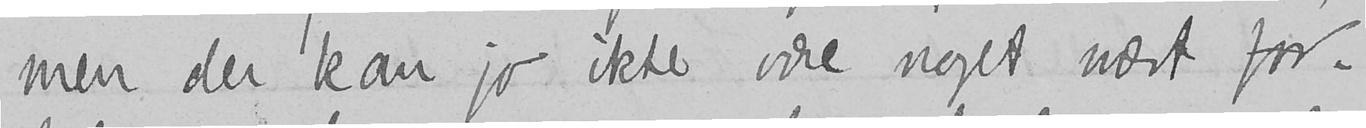men der kan jo ikke være noget nært for-
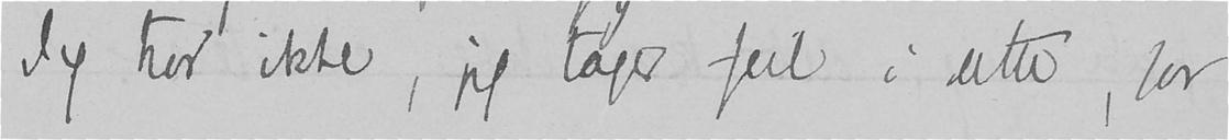Jeg tror ikke, jeg tager feil i dette, for
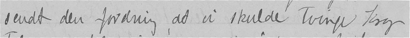sendt den fordring, at vi skulde tvinge Krog
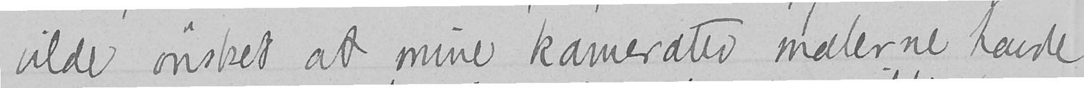vilde ønsket at mine kamerater malerne havde
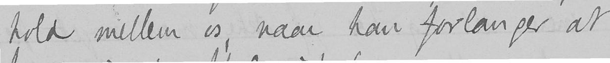hold mellem os, naar han forlanger at
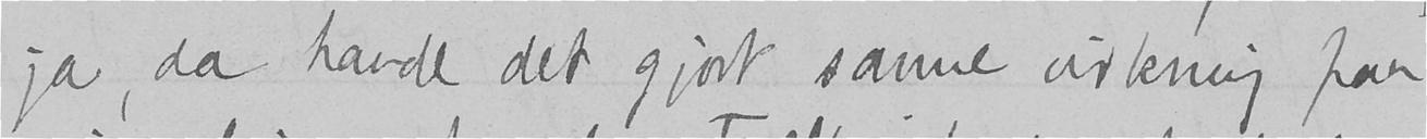ja, da havde det gjort samme virkning paa
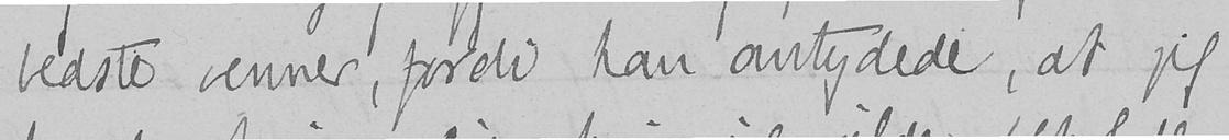bedste venner, fordi han antydede, at jeg
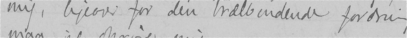mig, ligeover for den trælbindende fordring
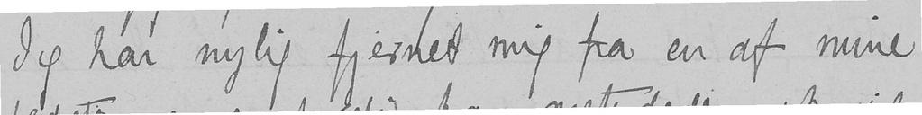Jeg har nylig fjernet mig fra en af mine
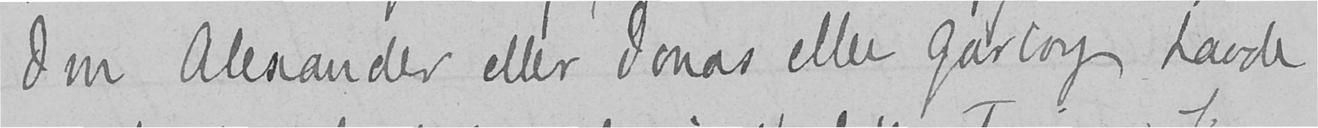Om Alexander eller Jonas eller Garbog havde
